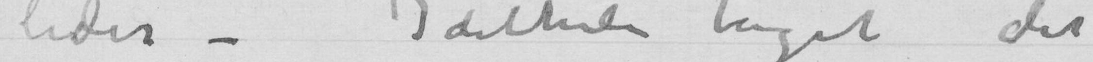lider – I dethele taget det
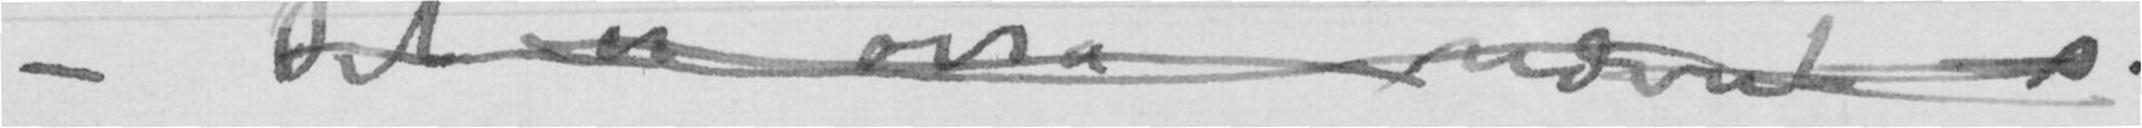– Det er osså nævnte s
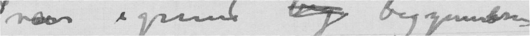var i grund beg begynnelsen
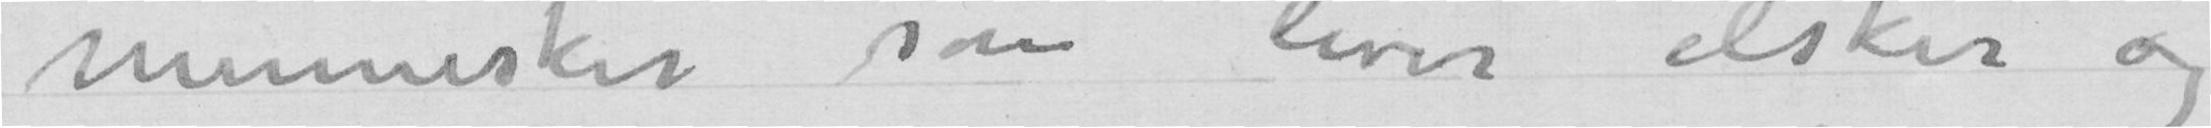mennesker som lever elsker og
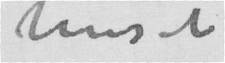huset
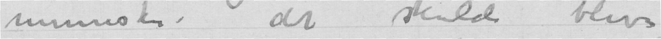mennesker, der skulde blive
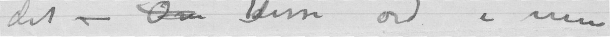det – O Disse ord i min
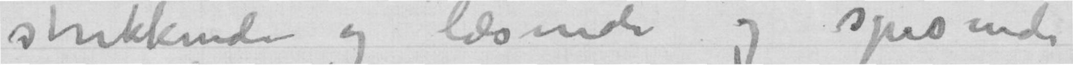strikkende og læsende og spisende
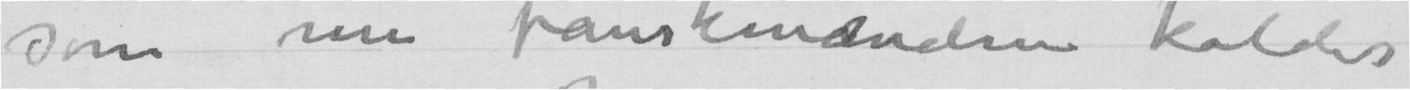som nu franskmænderne kalder
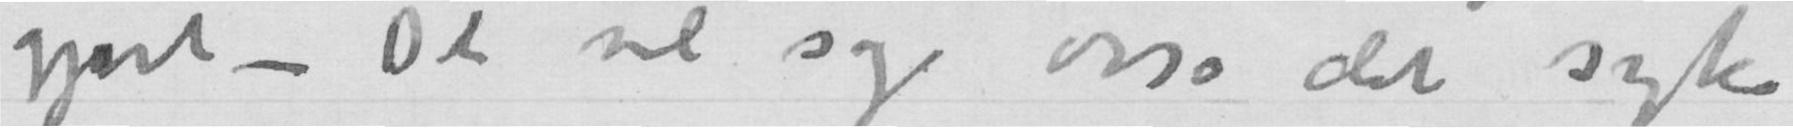gjort – Det vil sige osså det syke
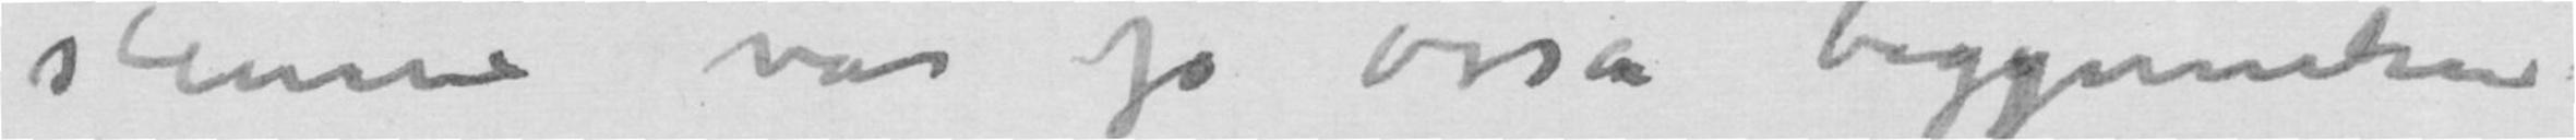stenerne var jo osså begynnelsen
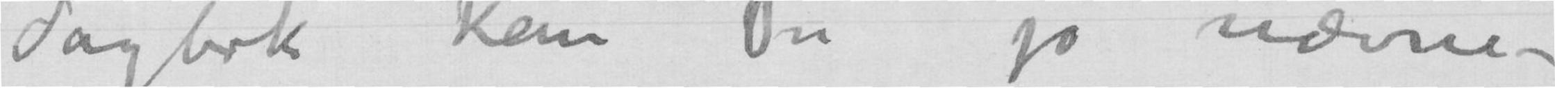dagbok kan Du jo nævne –
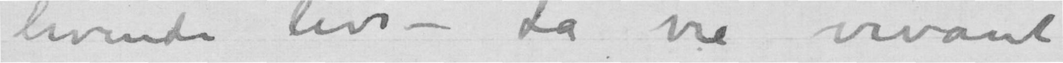levende liv – La vie vivant
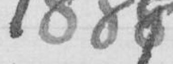1889
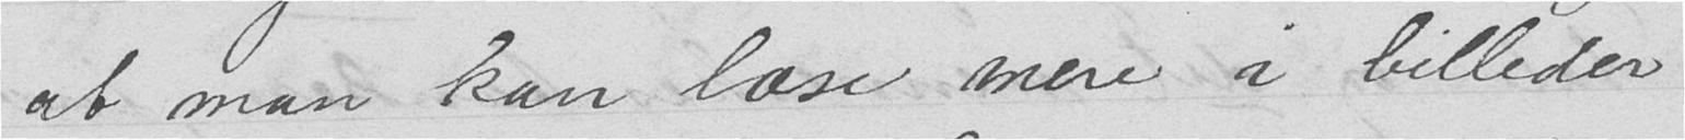at man kan læse mere i billeder
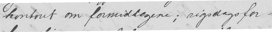kontoret om formiddagene; rigsdagsfor-
